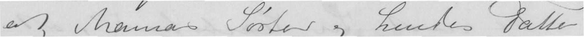at Mamas Søster og hendes Datter
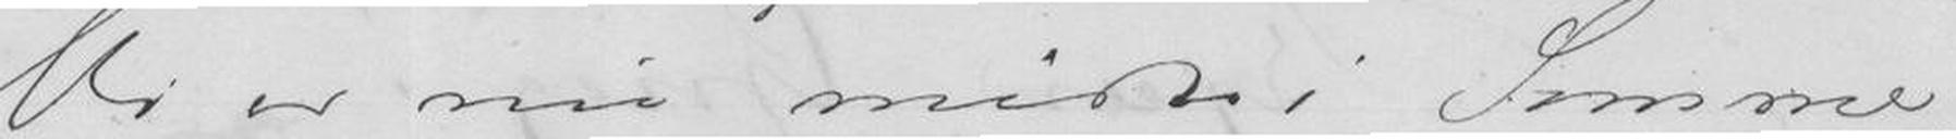Vi er nu midt i Somme-
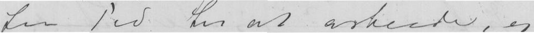faa Tid til at arbeide, og
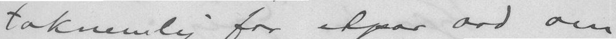taknemlig for etpar ord om
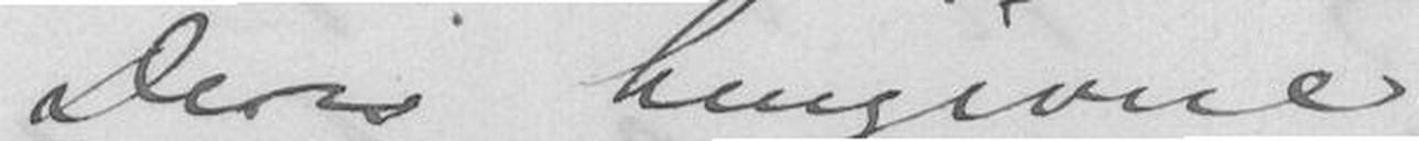Deres hengivne
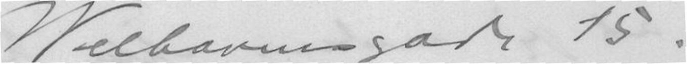Welhavensgade 15.
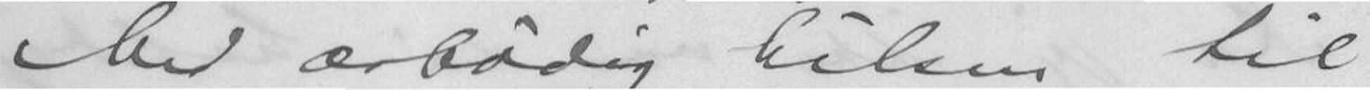Med ærbødig hilsen til
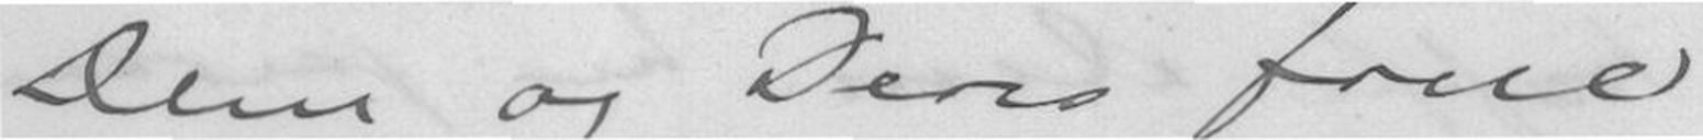Dem og Deres frue
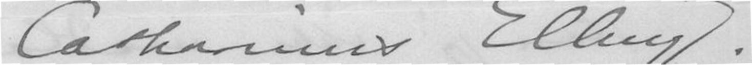Catharinus Elling
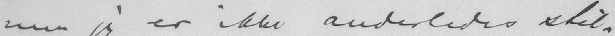men jeg er ikke anderledes stil-
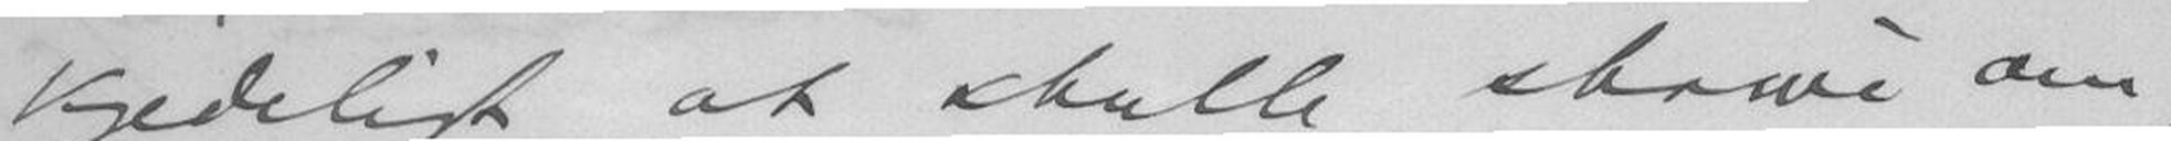kjedeligt at skulle skrive om
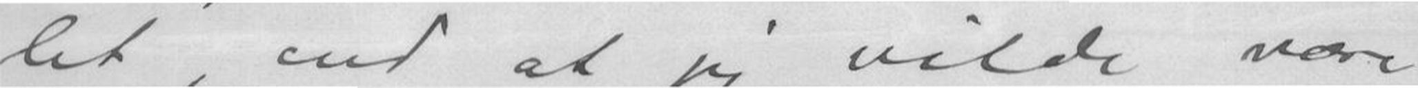let, end at jeg vilde være
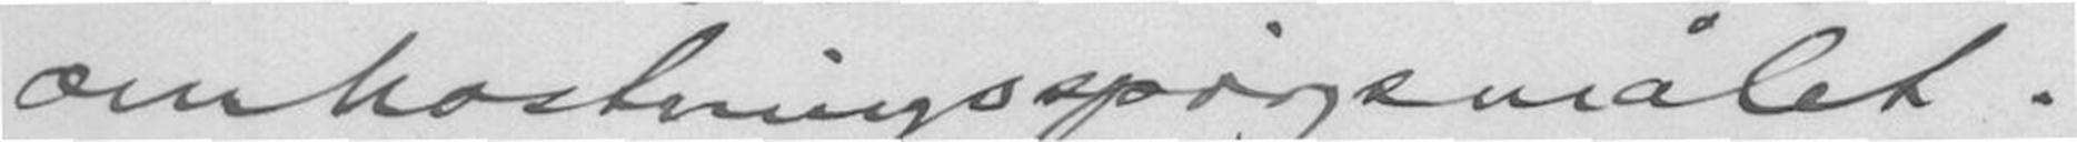omkostningsspørgsmålet.
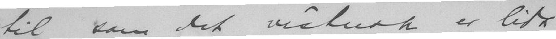til, som det vistnok er lidt
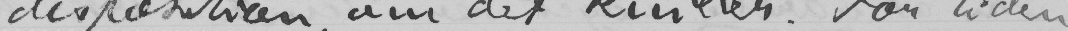disposistion, om det kniber. For tiden
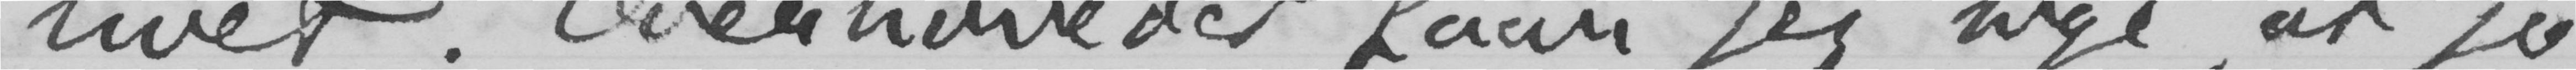livet. Overhovedet faar jeg sige, at jo
